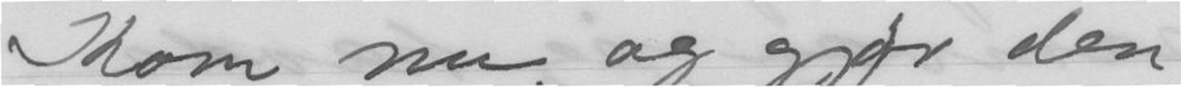Kom nu og gjør den
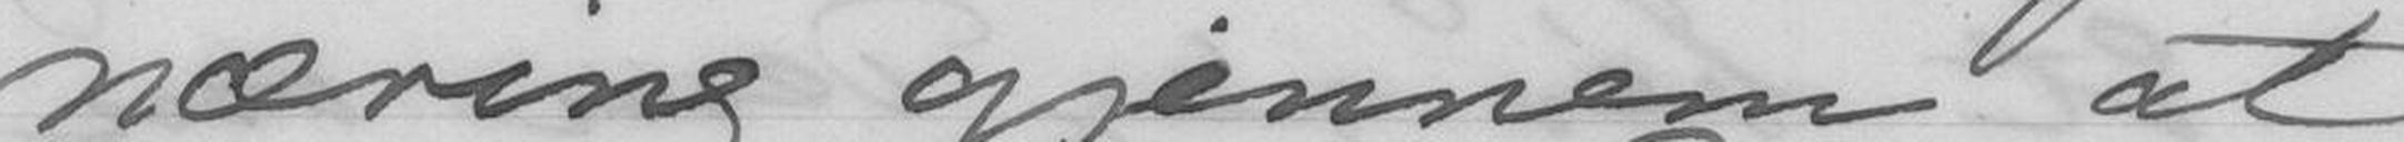næring gjennem at
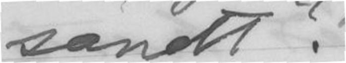sandt?
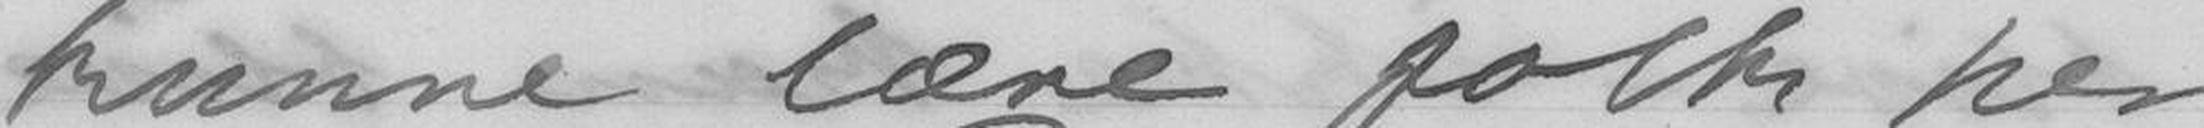kunne lære folk her
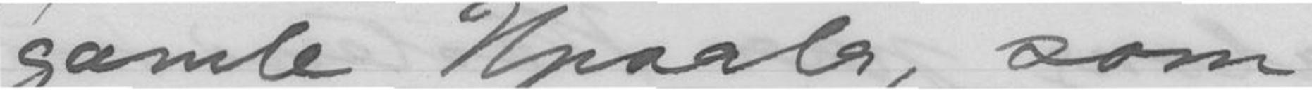gamle Upsala, som
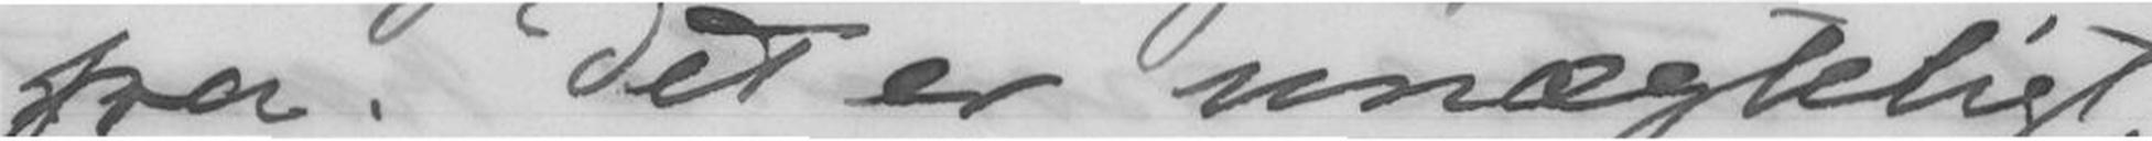fra. Det er unægteligt,
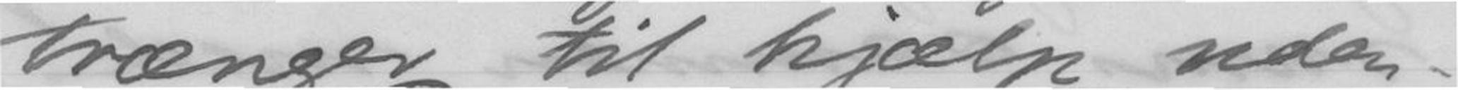trænger til hjælp uden-
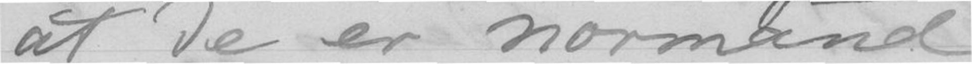at De er normænd.
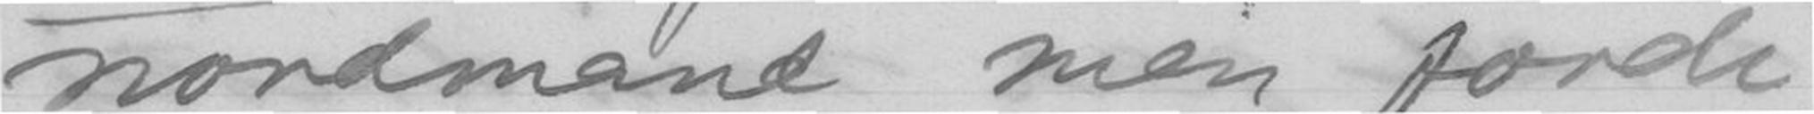nordmand men fordi
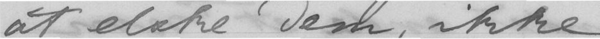at elske Dem, ikke
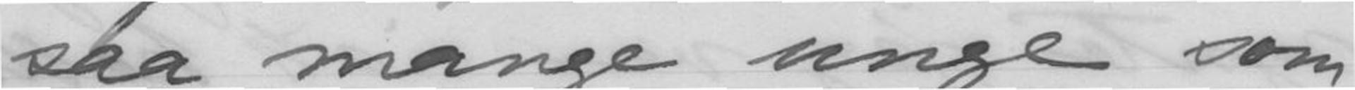saa mange unge som
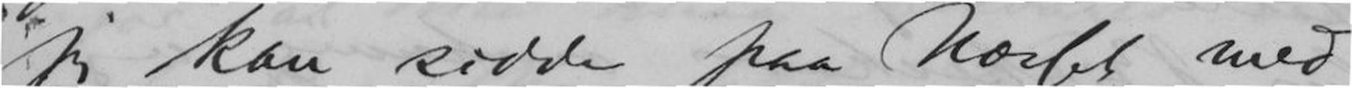jeg kan sidde paa Næsset med
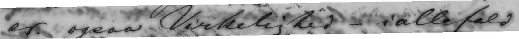er ogsaa Virkelighed - iallefald
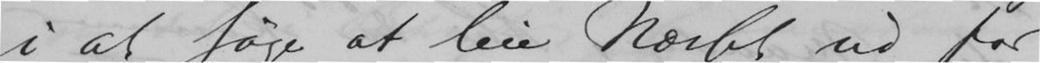i at søge at leie Næsset ud for
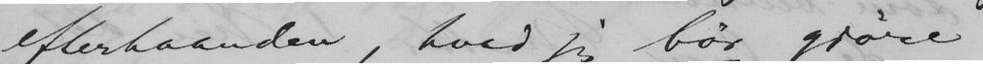efterhaanden, hvad jeg bør gjøre.
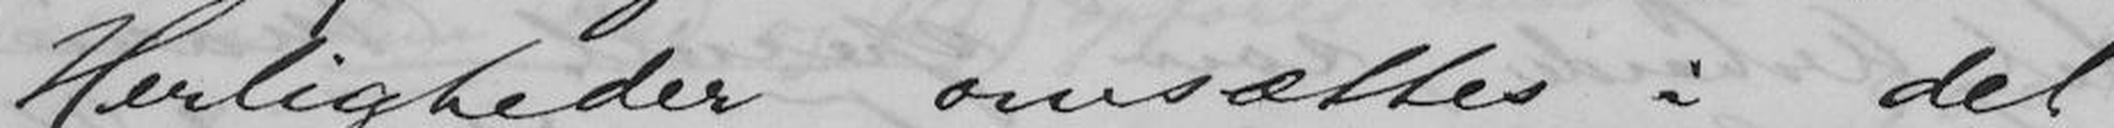Herligheder omsættes i det
